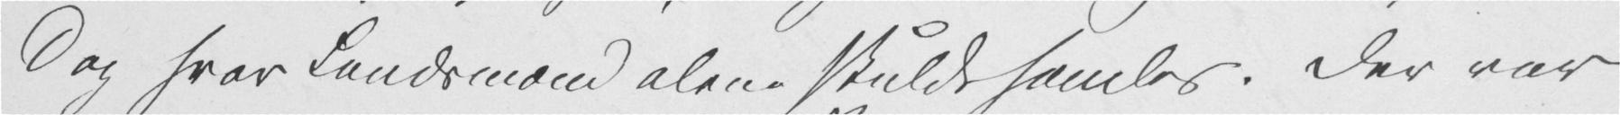Dag hvor Landsmænd alene skulde samles. Der var
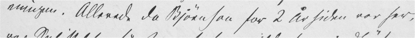eningen. Allerede da Bjørnson for 2 År siden var her,
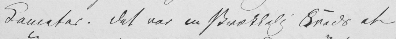Kanceter. Det var en skrækkelig Kreds at
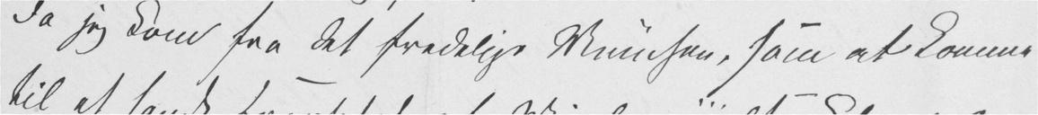da jeg kom fra det fredelige München, som at komme
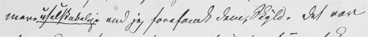mere uselskabelige end jeg forefandt dem, Skyld. Det var
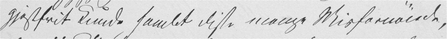gjestfrit kunde samlet disse mange Misfornøiede,
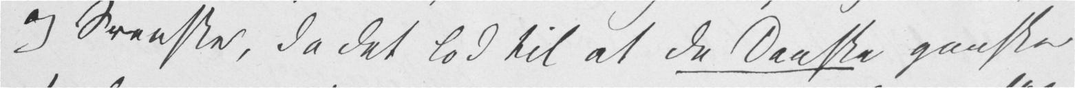og Svenske, da det lod til at de Danske ganske
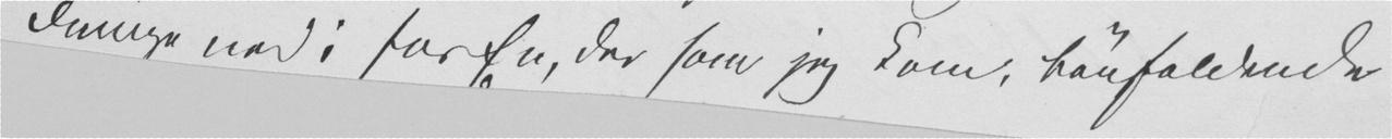dumpe ned i for En, der som jeg kom, bønfaldende
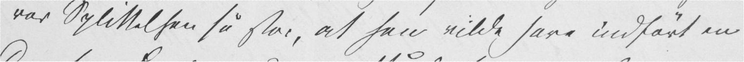var Splittelsen så stor, at han vilde have indført en
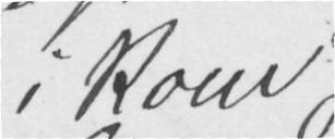i Rom
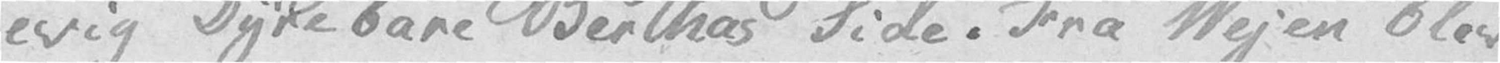evig Dyrebare Berthas Side. Fra Vejen blev
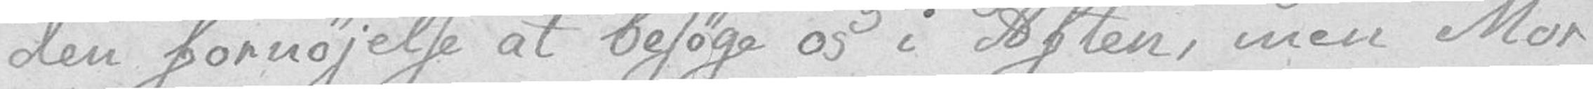den fornøjelse at besøge os i Aften, men Mor
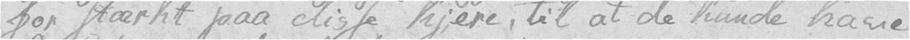for stærkt paa disse kjere, til at de kunde have
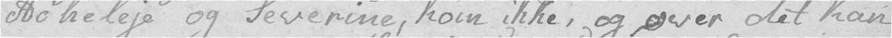Acheleje og Severine, kom ikke, og over det kan
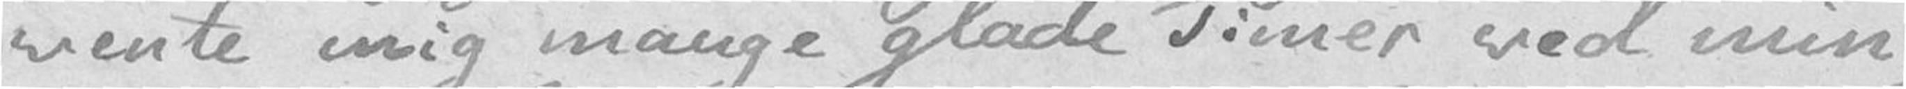vente mig mange glade Timer ved min
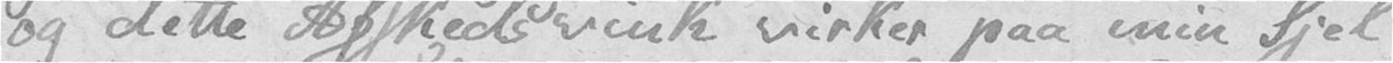og dette Afskedsvink virker paa min Sjel
